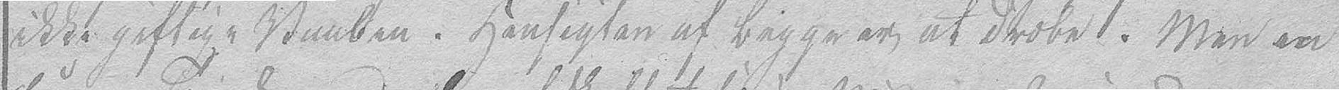ikke giftige Vaaben. Hensigten af begge er at dræbe. Men en
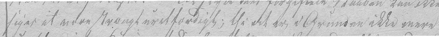siges at være strængt uretfærdigt; thi det er i Grunden ikke mere
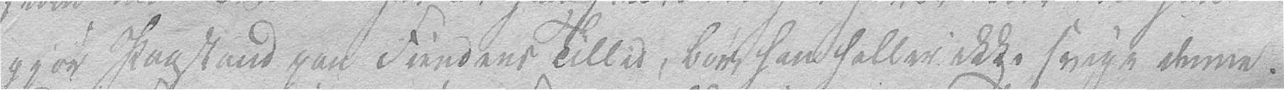gjør Paastand paa Fiendens Tillid, bør han heller ikke svige denne.
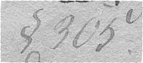§ 305.
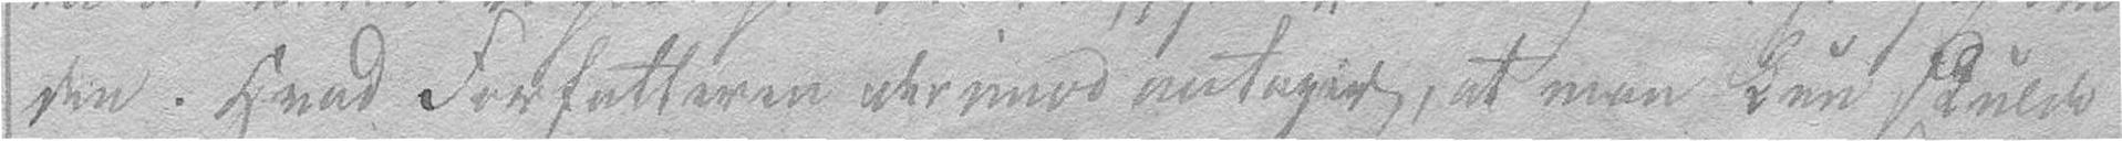den. Hvad Forfatteren derimod antager, at man kun skulde
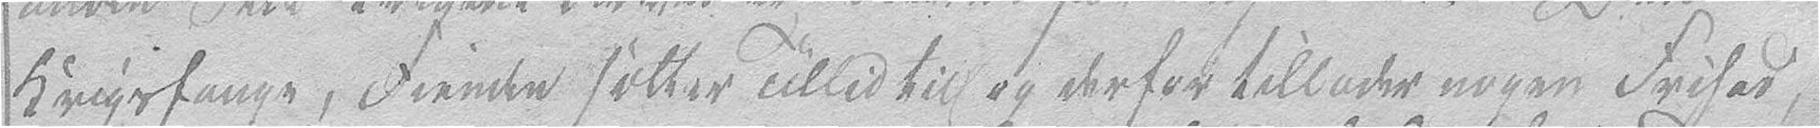Krigsfange, Fienden sætter Tillid til og derfor tillader nogen Frihed,
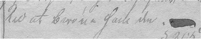til at berøve ham den. –
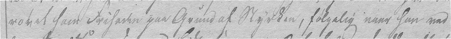røvet ham Friheden paa Grund af Styrken, følgelig naar han ved
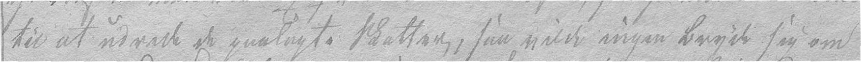til at udrede de paalagte Skatter, saa vilde ingen bryde sig om
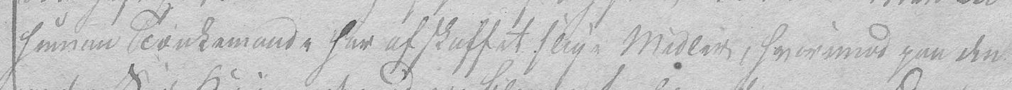human Tænkemande har afskaffet slige Midler, hvorimod paa den
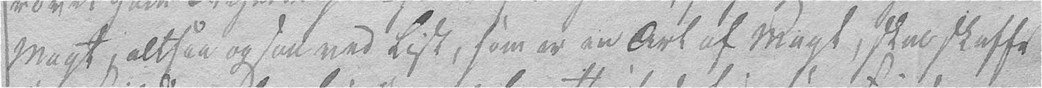Magt, altsaa ogsaa ved List, som er en Art af Magt, skal skaffe
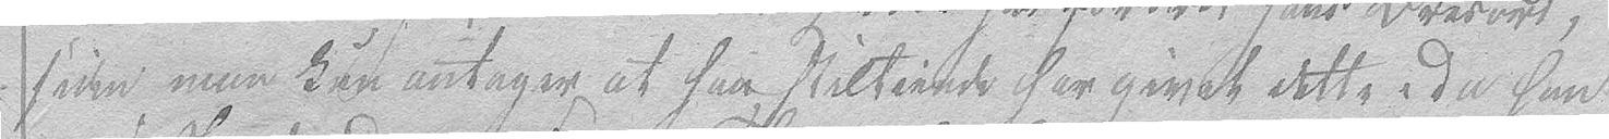siden man kun antager at han stiltiende har givet dette. Da han
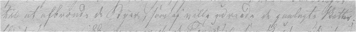til at afbrænde de Byer, som ey ville udrede de paalagte Skatter;
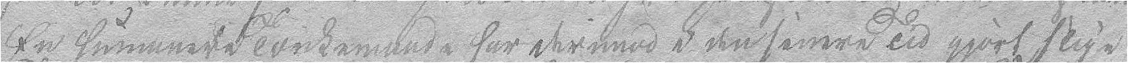En humanere Tænkemende har derimod i den senere Tid gjort slige
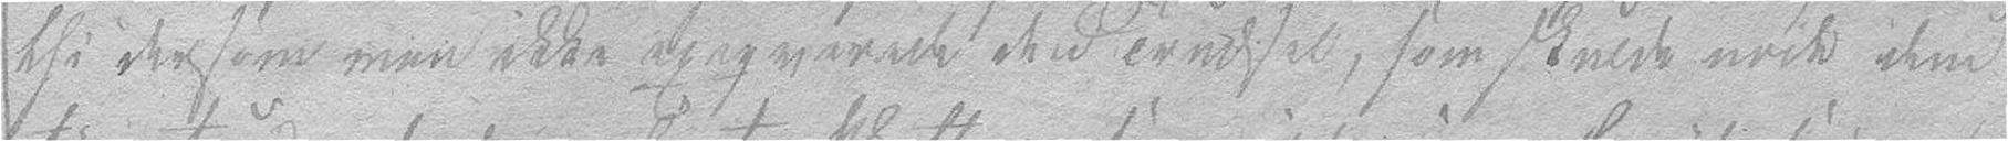thi dersom man ikke exeqverede den Trudsel, som skulde nøde dem
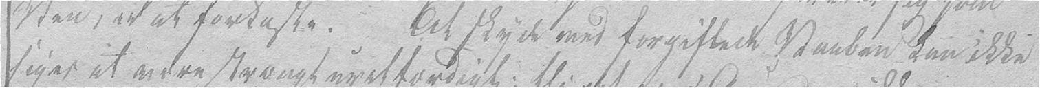Ven, er at forkaste. At skyde med forgiftede Vaaben kan ikke
